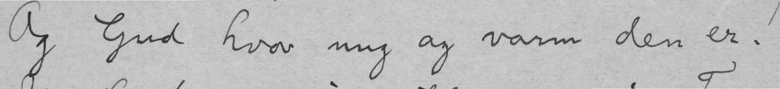Og Gud hvor ung og varm den er!
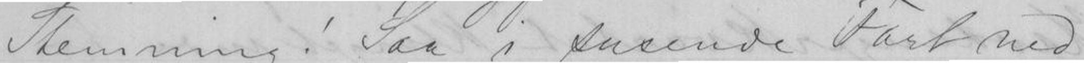Stemning! Saa i susende Fart ned
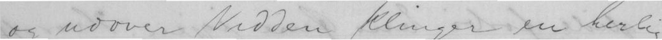og udover Vidden klinger en herlig
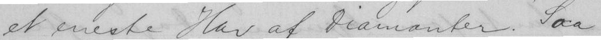et eneste Hav af Diamanter. Saa
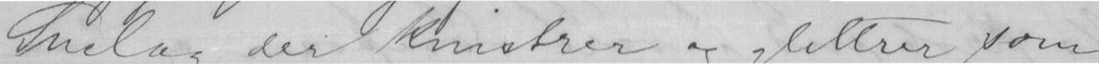Snelag der knistrer og glittrer som
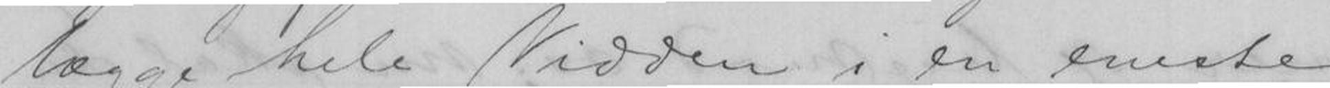lægge hele Vidden i en eneste
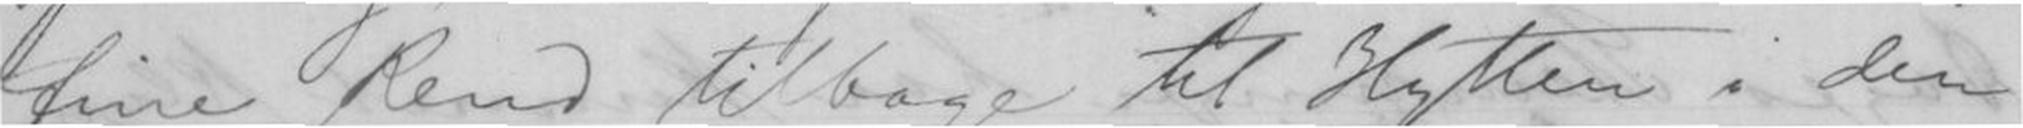fine Rend tilbage til Hytten i den
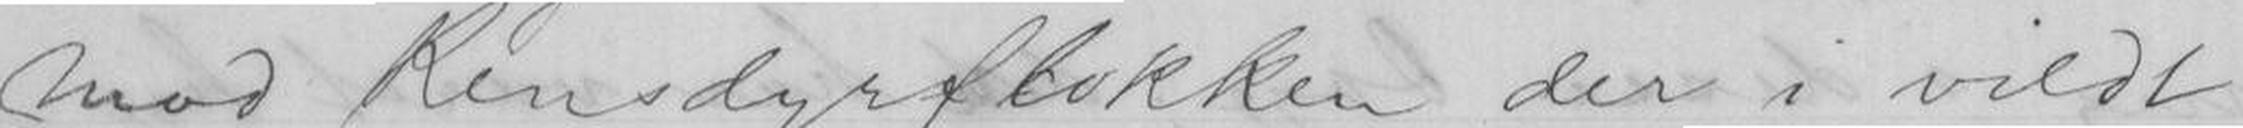mod Rensdyrflokken der i vildt
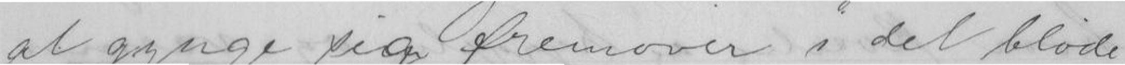at gynge sig fremover i det bløde
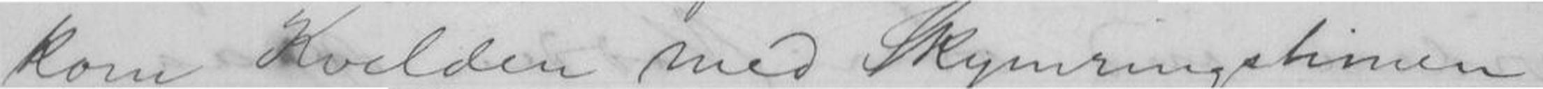kom Kvelden med Skymringstimen
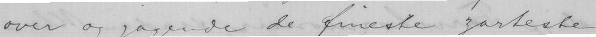over og jagende de fineste zarteste
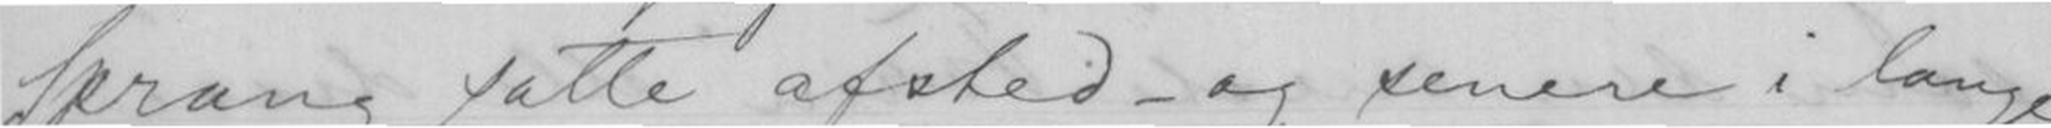Sprang satte afsted - og senere i lange
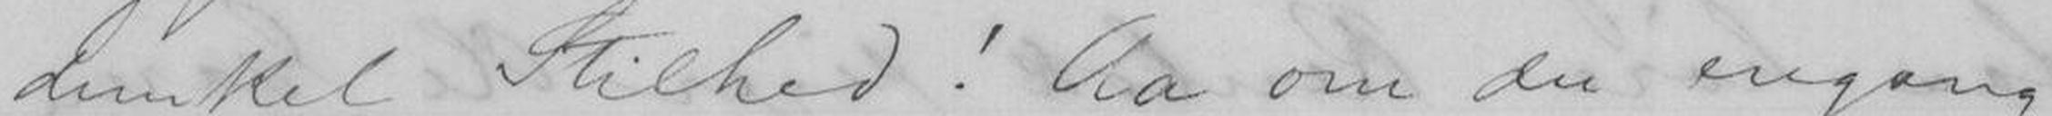dunkel Stilhed! Aa, om du engang
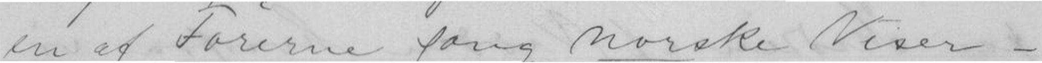en af Førerne sang norske Viser -
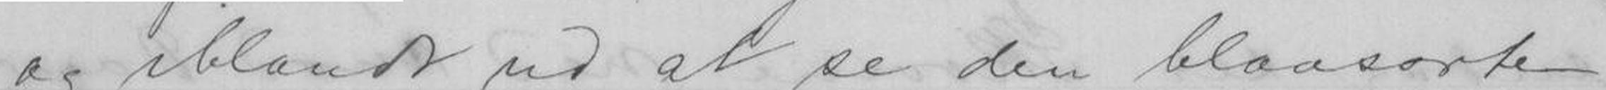og iblandt ud at se den blaasorte
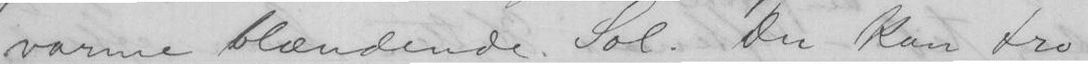varme blændende Sol. Du kan tro
